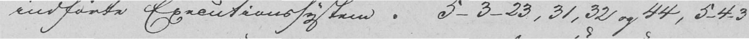indførte Executionssystem. 5-3-23, 31, 32 og 44, 5-4-3
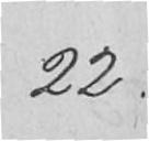22.
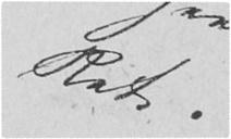Ret.
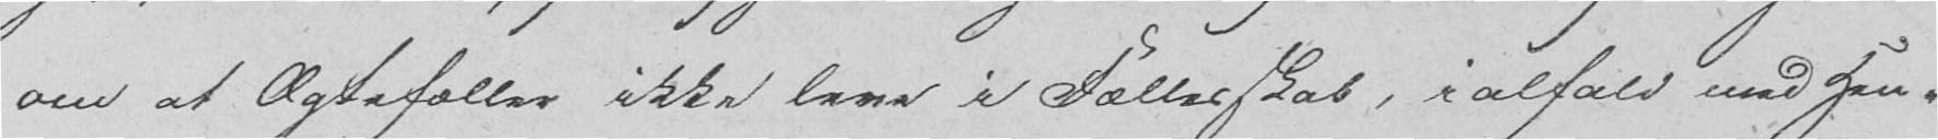om at Ægtefæller ikke leve i Fællesskab, i alfald med Hen-
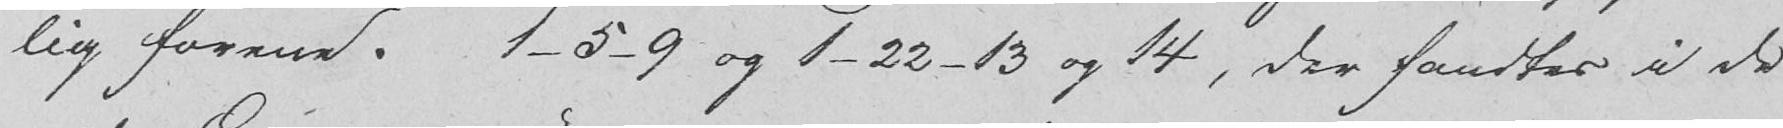lig forene. 1-5-9 og 1-22-13 og 14, der fandtes i de
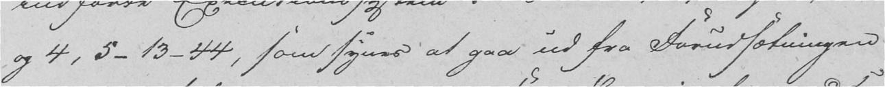og 4, 5-13-44, som synes at gaa ud fra Forudsætningen
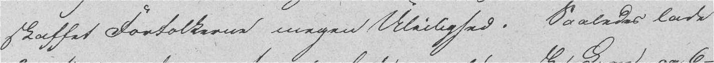skaffet Fortolkerne megen Uleilighed. Saaledes lade
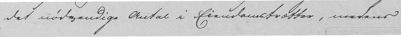det nødvendige Antal i Eiendomstrætter, medens
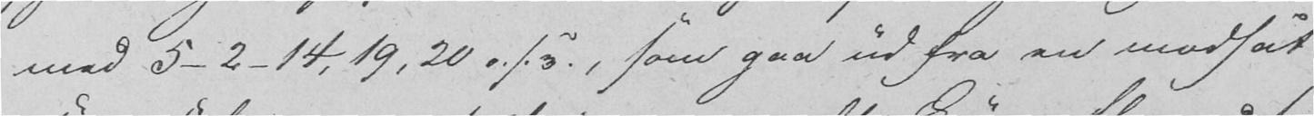med 5-2-14, 19, 20 o. s. v., som gaa ud fra en modsat
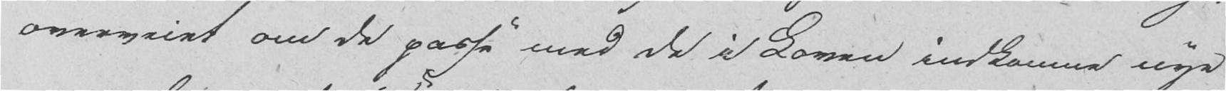overveiet om de passe med de i Loven indkomne nye
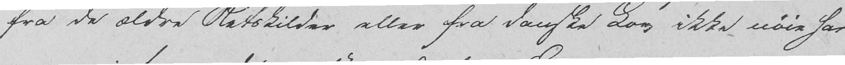fra de ældre Retskilder eller fra danske Lov ikke nøie har
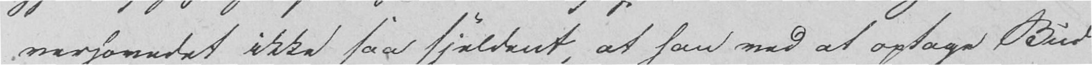verhovedet ikke saa sjeldent, at han med at optage Bud
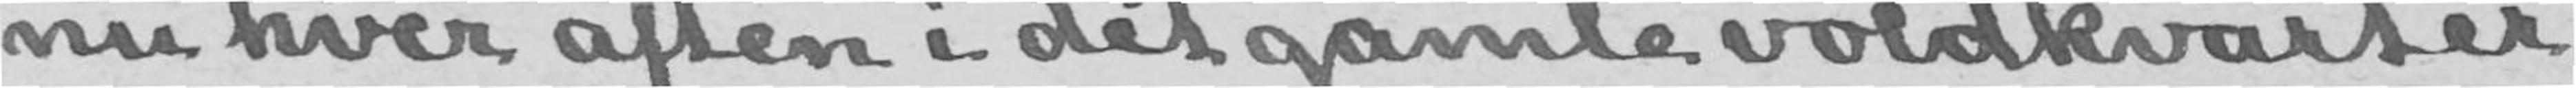nu hver aften i det gamle voldkvartér
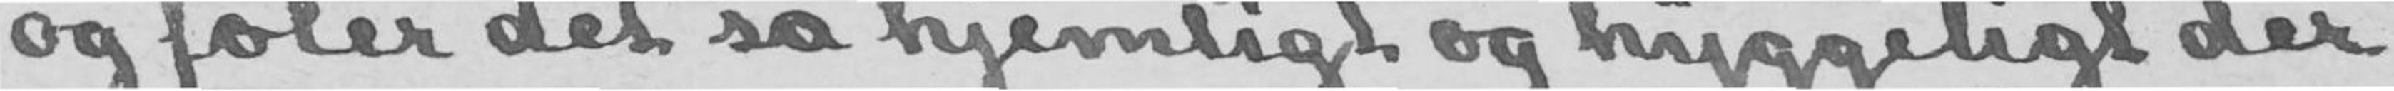og føler det så hjemligt og hyggeligt der
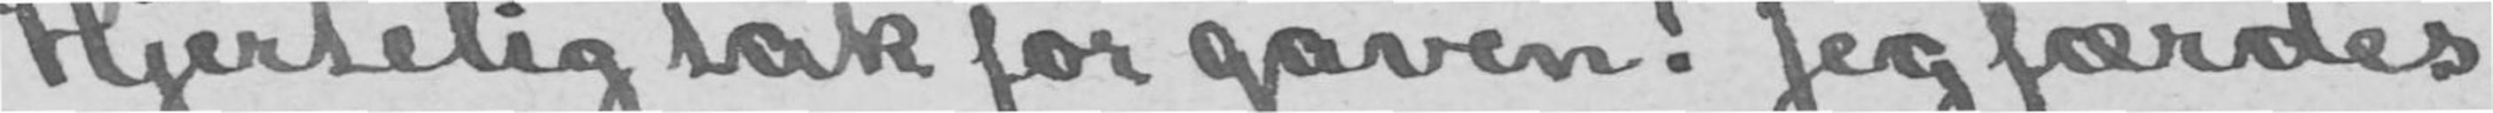Hjertelig tak for gaven! Jeg færdes
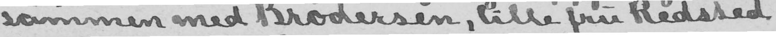sammen med Brodersen, lille fru Redsted
